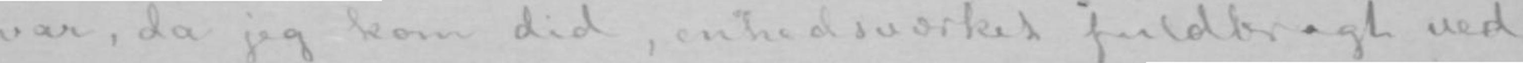var, da jeg kom did, enhedsværket fuldbragt ved
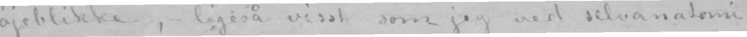øjeblikke,- ligeså visst som jeg ved selvanatomi
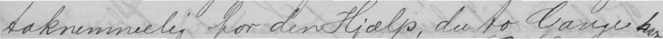taknemmlig for den Hjælp, du to Gange har
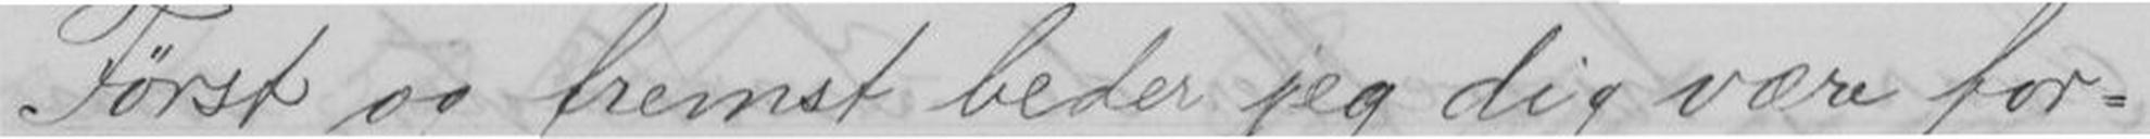Først og fremst beder jeg dig være for-
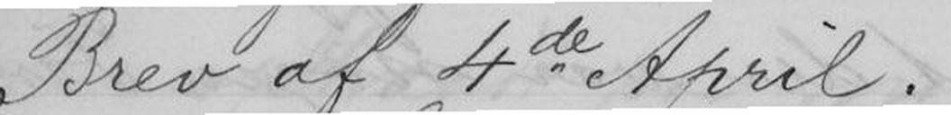Brev af 4de April.
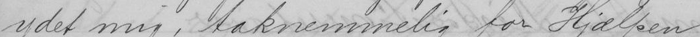ydet mig, taknemmelig for Hjælpen
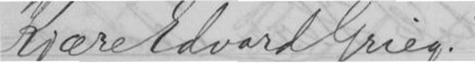Kjære Edvard Grieg!
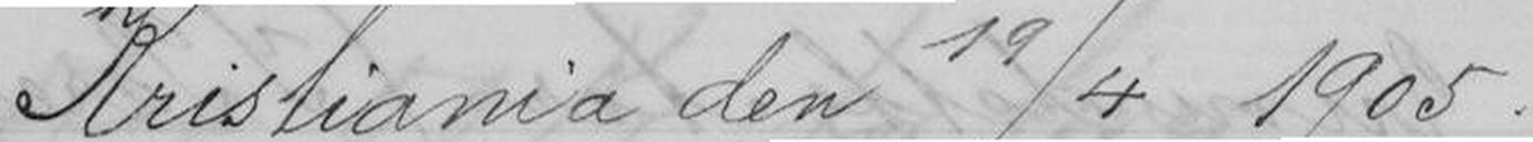Kristiania den 19/4 1905.
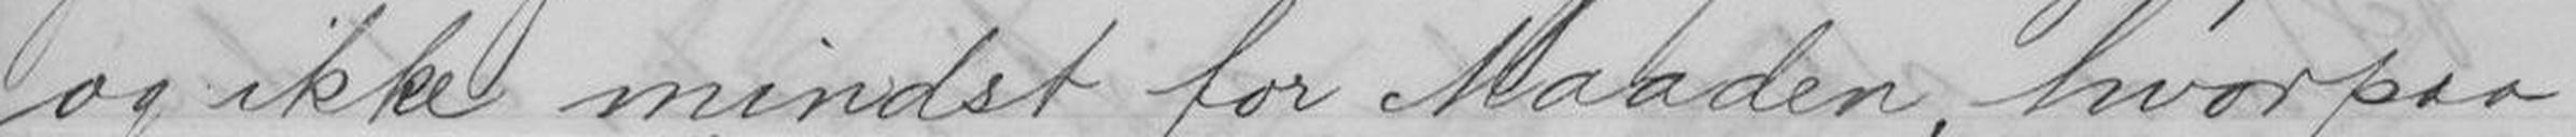og ikke mindst for Maaden, hvorpaa
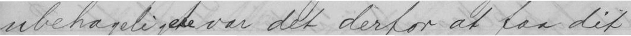ubehageligere var det derfor at faa dit
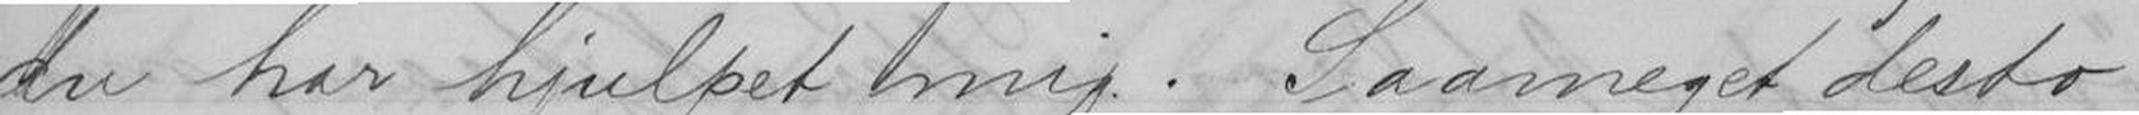du har hjulpet mig. Saameget desto
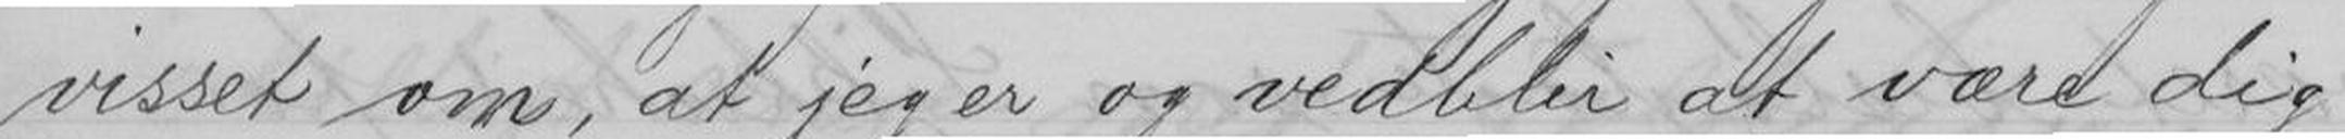visset om, at jeg er og vedblir at være dig
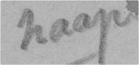haap
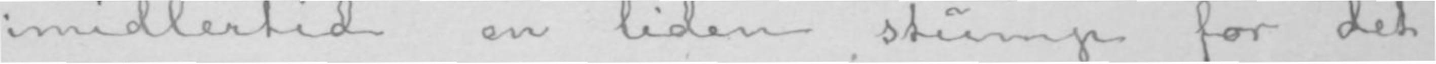imidlertid en liden stump for det
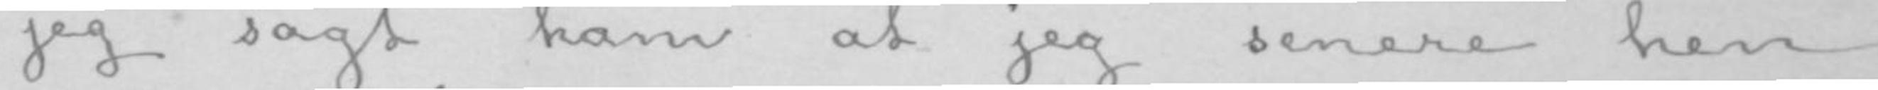jeg sagt ham at jeg senere hen
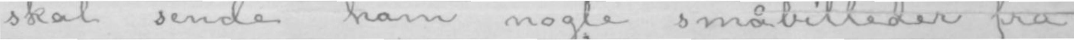skal sende ham nogle småbilleder fra
